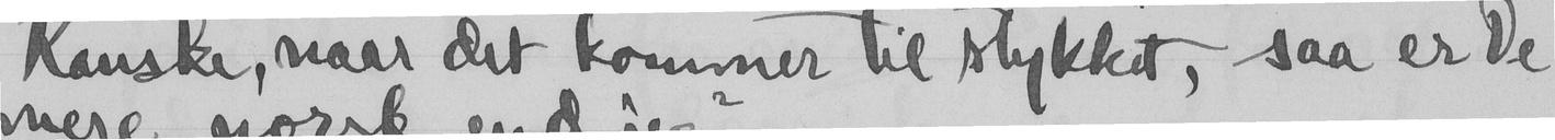Kanske, naar det kommer til stykket, - saa er De
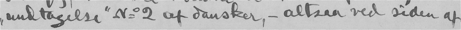"undtagelse" No 2 af dansker, - altsaa ved siden af
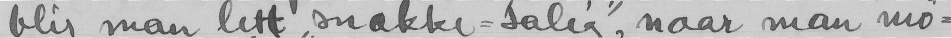blir man lett snakke-salig, naar man mø-
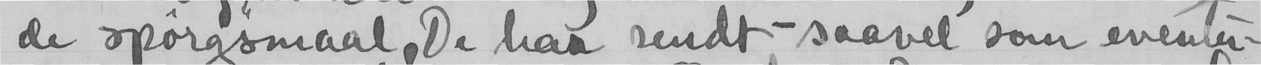de spørgsmaal De har sendt - saavel som eventu-
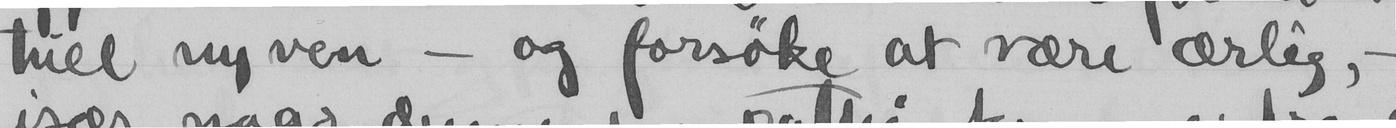tuel ny ven - og forsøke at være ærlig, -
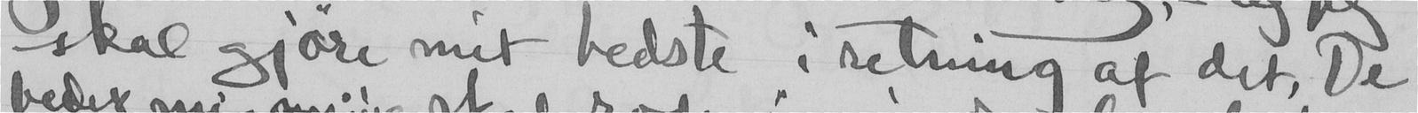skal gjøre mit bedste i retning af det, De
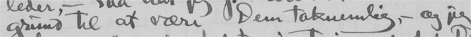grud til at være Dem taknemlig, - og jeg
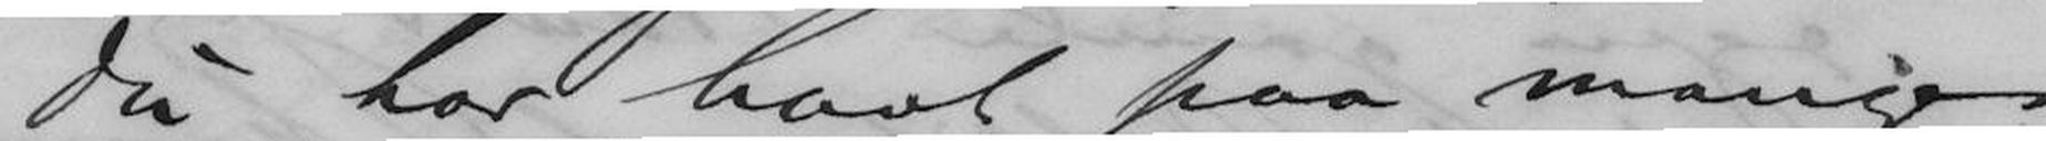du har havt paa mange
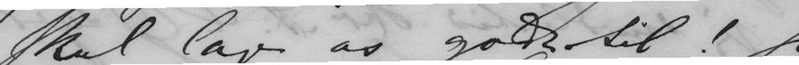skal lage os godt til! Jeg
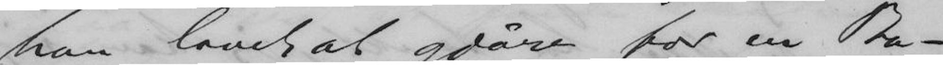han lovet at gjøre for en Ba-
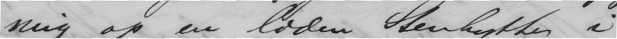mig op en liden Stenhytte i
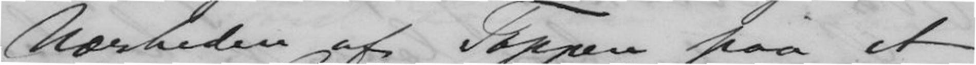Nærheden af Toppen paa et
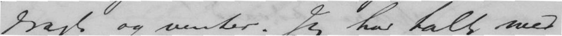dragt og venter. Jeg har talt med
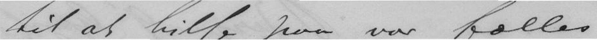til at hilse paa vor fælles
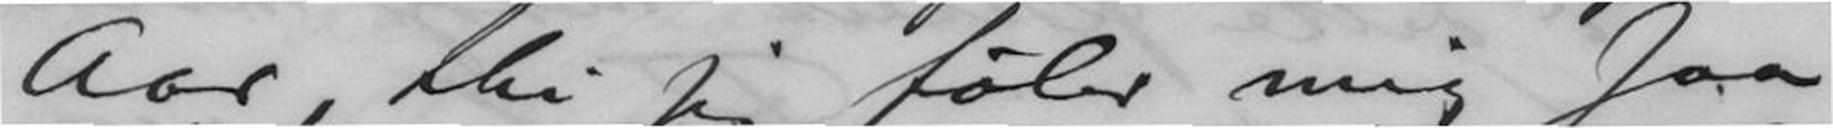Aar, thi jeg føler mig saa
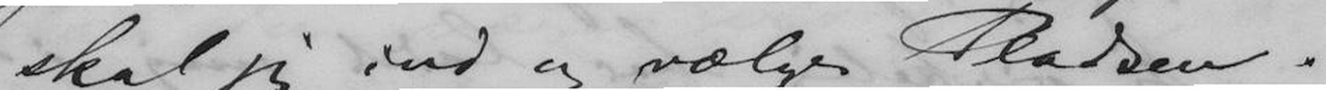skal jeg ind og vælge Pladsen.
